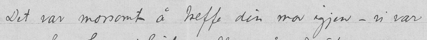Det var morsomt å treffe din mor igjen – vi var
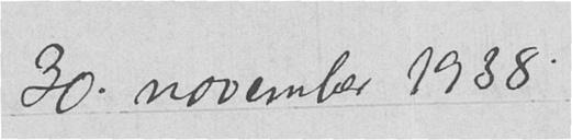30. november 1938.
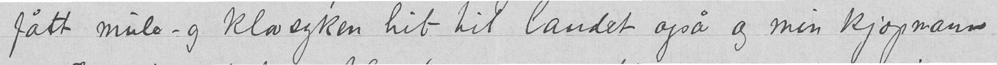fått mule- og klovsyken hit til landet også og min kjøpmann
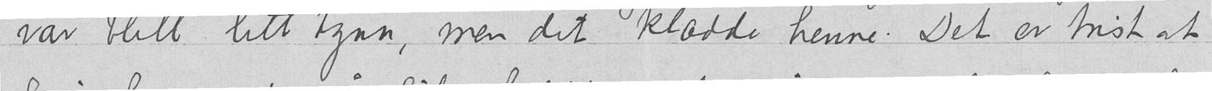var blitt litt tynn, men det klædde henne. Det er trist at
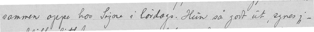sammen oppe hos Signe i lørdags. Hun så godt ut, synes jeg –
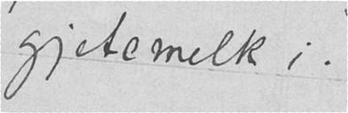gjetemelk i.
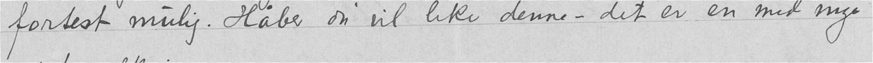fortest mulig. Håber du vil like denne – det er en med mye
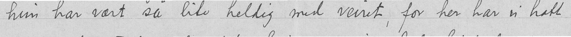hun har vært så lite heldig med veiret, for her har vi hatt
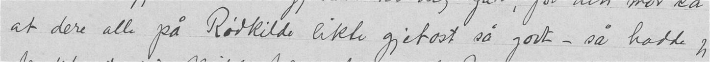at dere alle på Rødkilde likte gjetost så godt – så hadde jeg
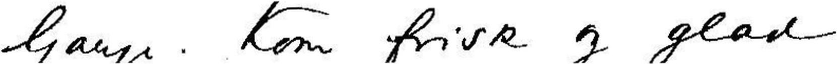Gange. Kom frisk og glad
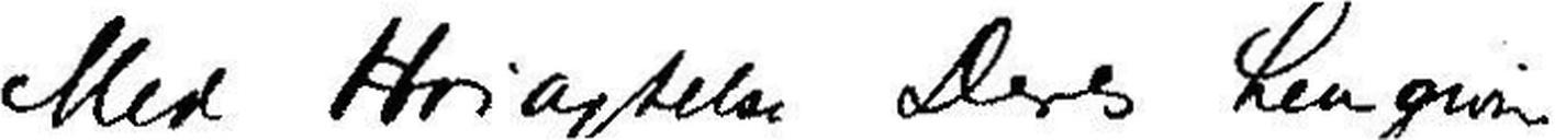Med Høiagtelse Deres hengivne
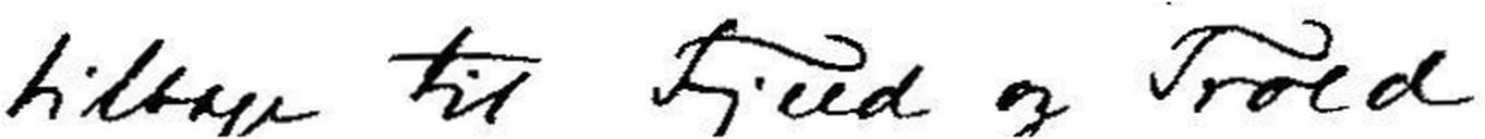tilbage til Fjeld og Trold
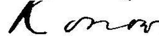Konow
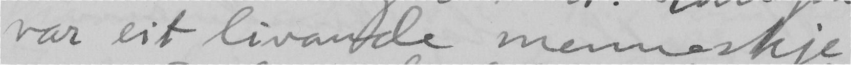var eit livande menneskje
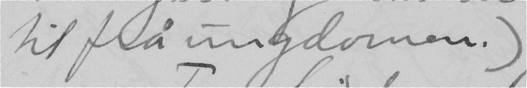til frå ungdomen.
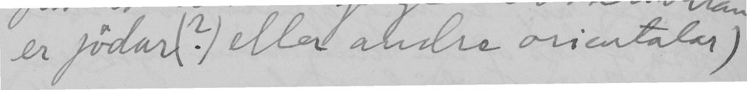er jödar (?) eller andre orientalar.
